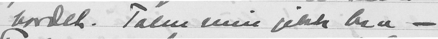bordet. Talen min gikk bra -
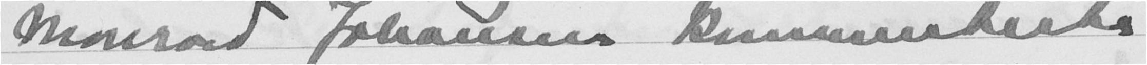Monrad Johansens kommenkiske
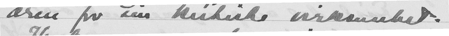æren for sin kritiske virksomhet.
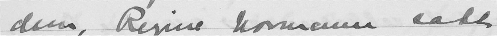dem, Regine Normann satt
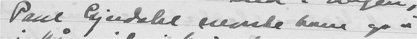Paul Gjerdahl nevnte ham også
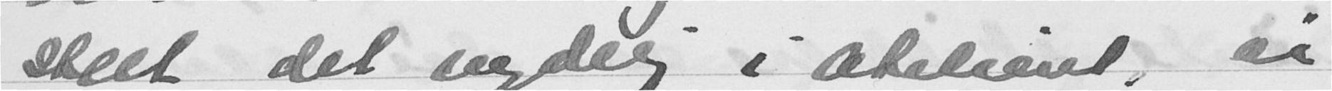stelt det nydelig i ateleriet, vi
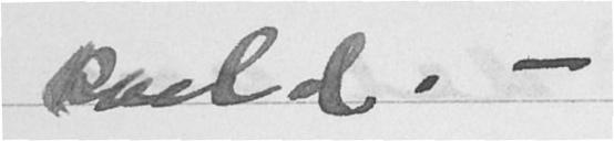kveld. -
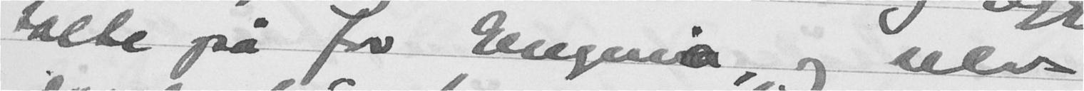talte på for Eugenia, og selv
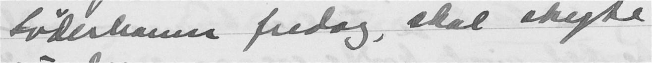Søderhamn fredag, skal skyte
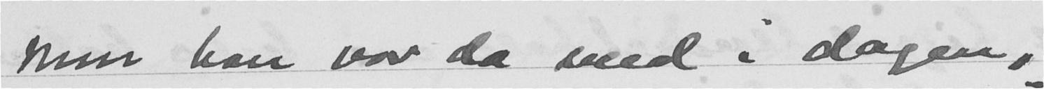Men han var da med i dagen,
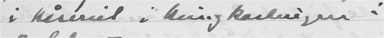i kåseriet i kringkastingen i
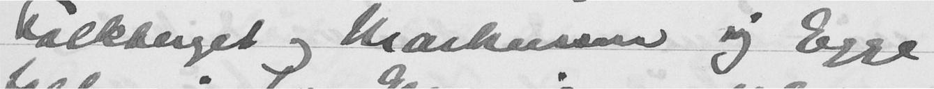Falkberget og Markussen og Egge
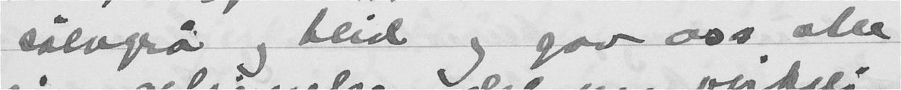sølvgrå og blid og gav oss alle
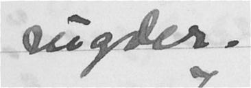rugder.
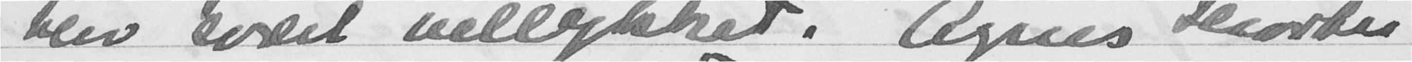blev svært vellykket. Agnes Hiorths
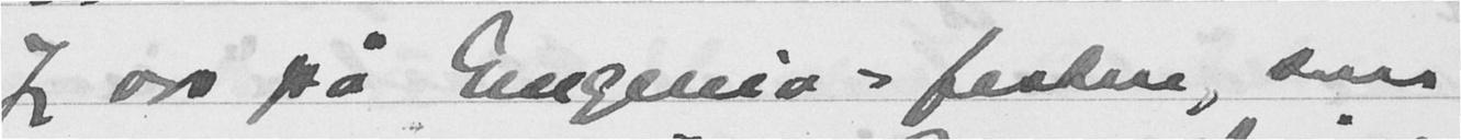Jeg var på Eugenia-festen, som
